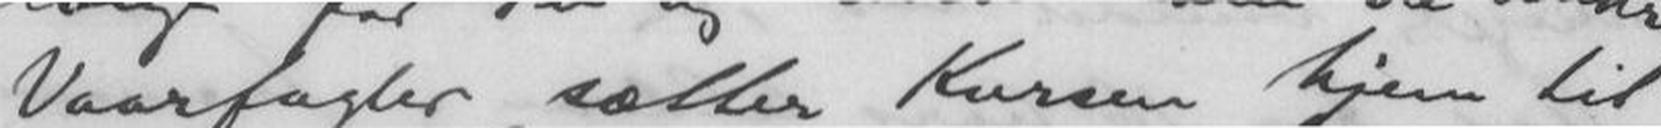Vaarfugler sætter Kursen hjem til
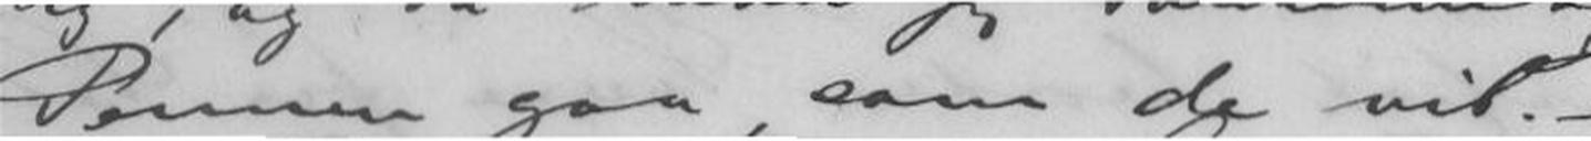Pennen gaa, som de vil. -
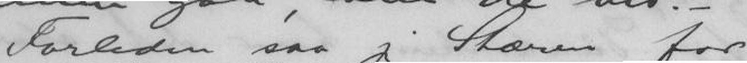Forleden saa jeg Stæren for
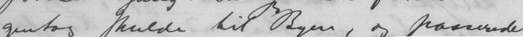gentog skulde til Byen, og passerede
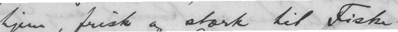hjem, frisk og stærk til Fiske-
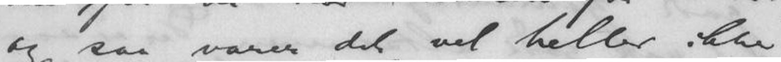og saa varer det vel heller ikke
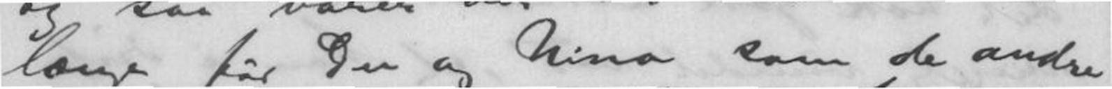længe før Du og Nina som de andre
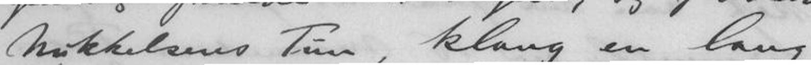Mikkelsens Tun, klang en lang
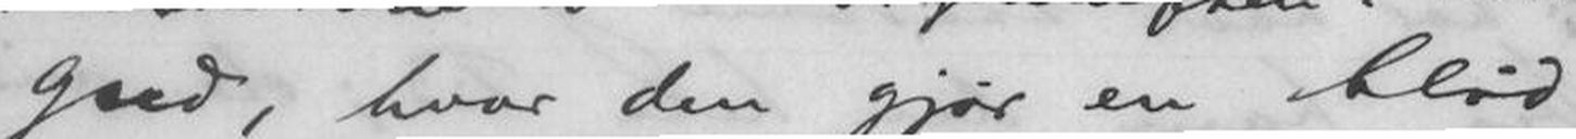gud, hvor den gjør en blød
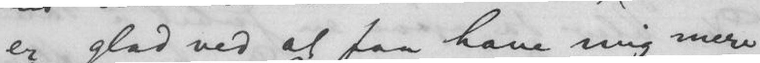er glad ved at faa have mig mere
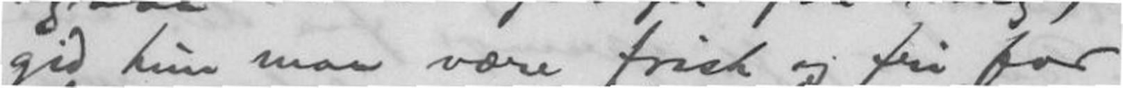gid hun maa være frisk og fri for
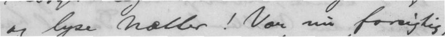og lyse Nætter! Vær nu forsigtig,
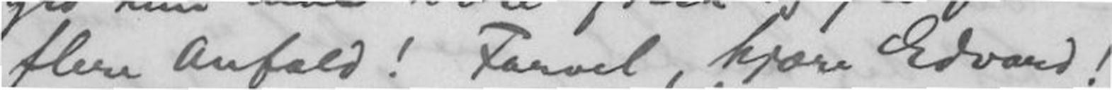flere Andfald! Farvel, kjære Edvard!
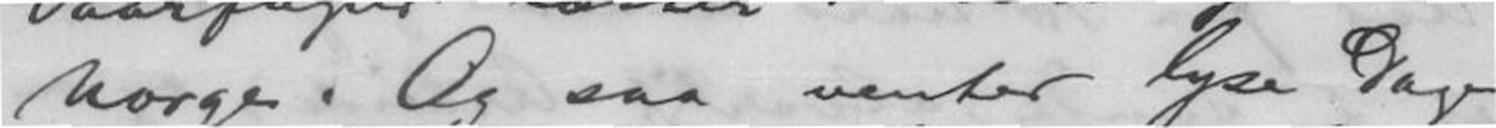Norge. Og saa venter lyse Dage
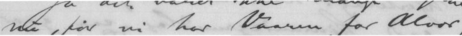nu, før vi har Vaaren for Alvor,
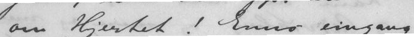om Hjertet! Enno eingang
